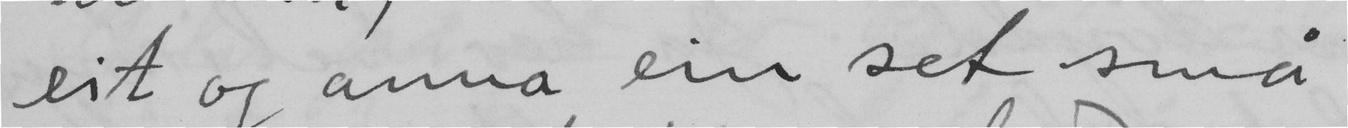eit og anna ein set små
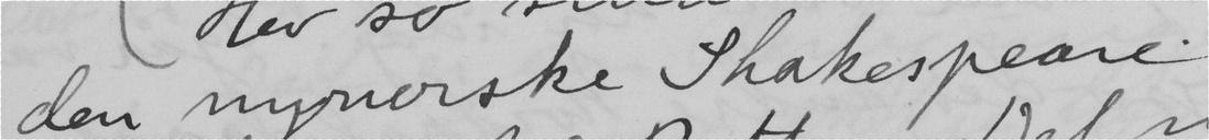den nynorske Shakespeare
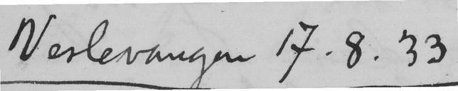Veslevangen 17.8.33
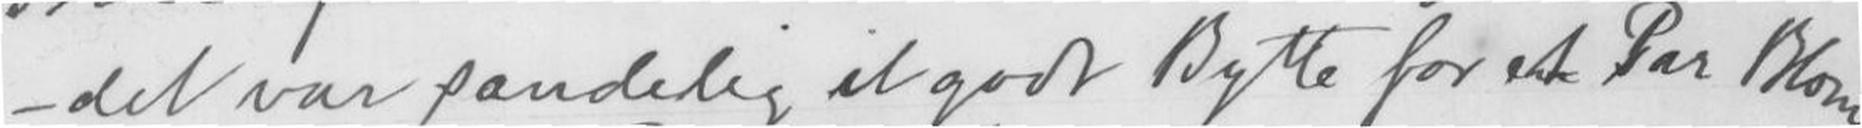- det var sandelig et godt Bytte for et Par Blom
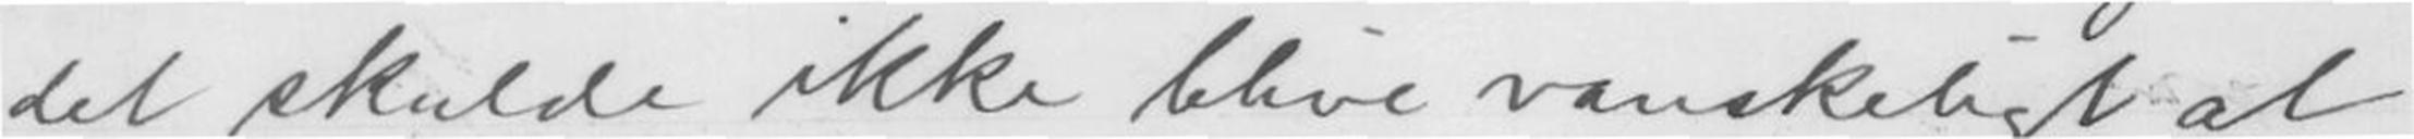det skulde ikke blive vanskeligt at
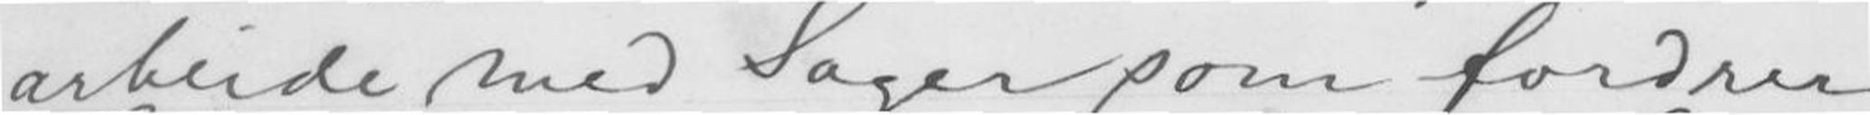arbeide med Sager som fordrer
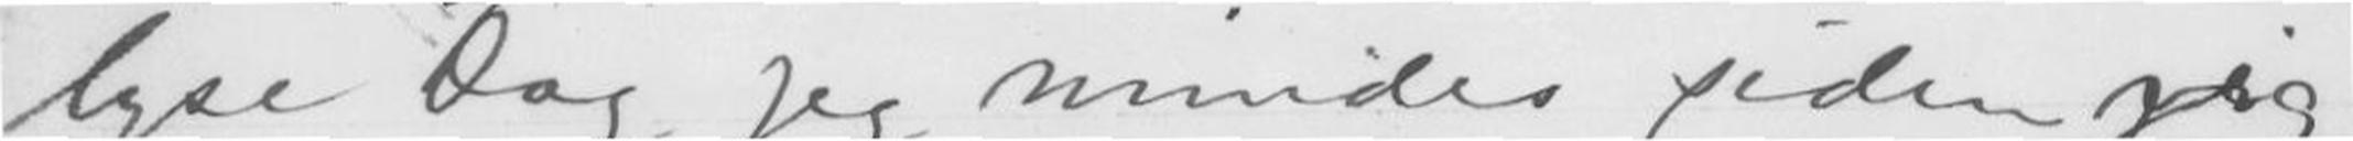lyse Dag jeg mindes siden jeg
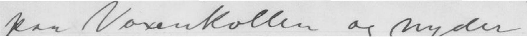paa Voxenkollen og nyder
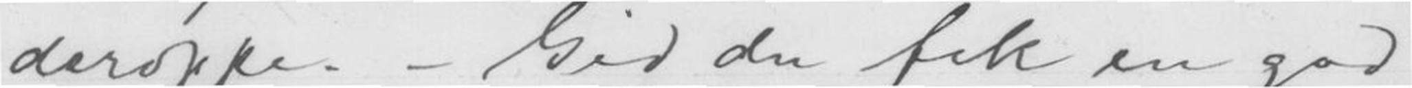deroppe. - Gid du fik en god
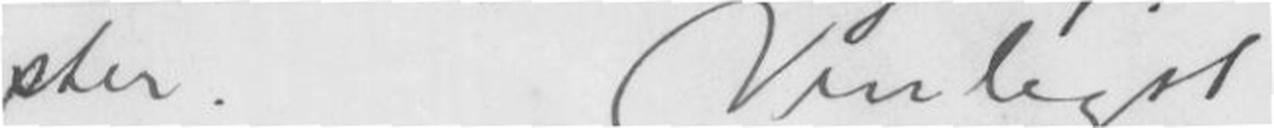ster. Venligst
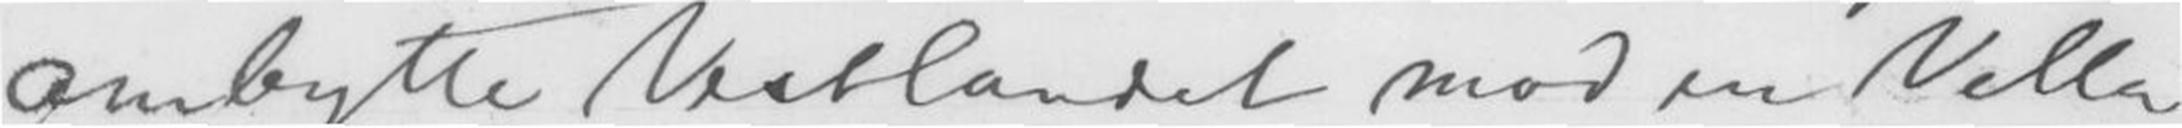ombytte Vestlandet mod en Villa
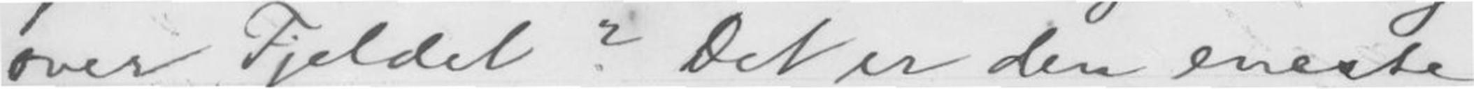over Fjeldet? Det er den eneste
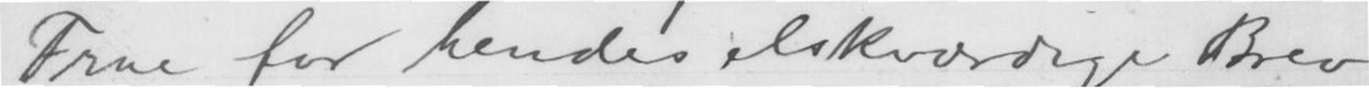Frue for hendes elskværdige Brev
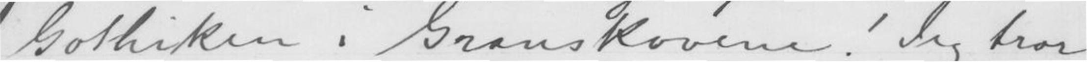Gothiken i Granskovene! Jeg tror
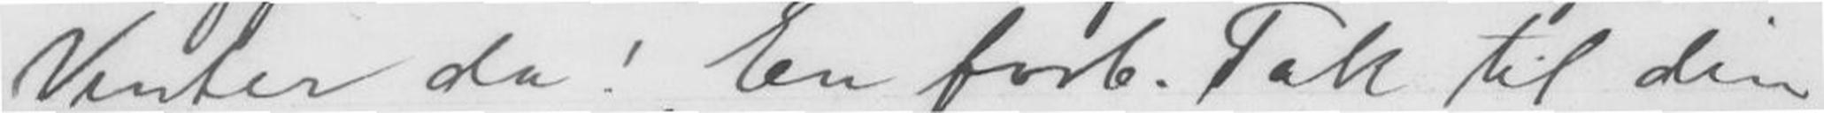Vinter da! En forb. Tak til din
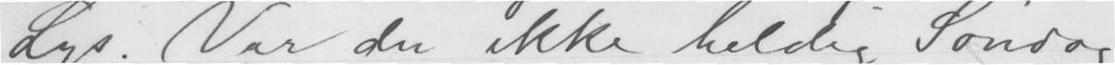Lys. Var du ikke heldig Søndag
